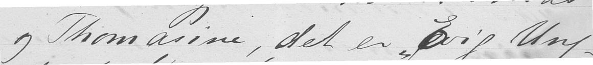og Thomasine, det er "Evig Ung-
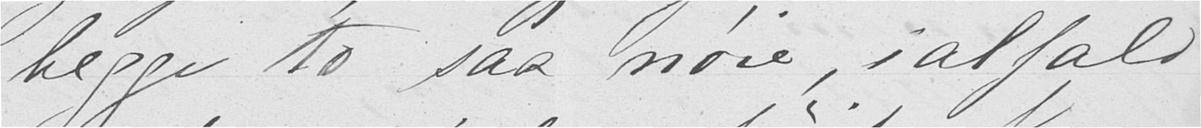begge to saa nøie, ialfald
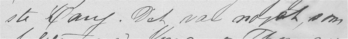ste Rang. Det var noget, som
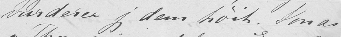vurderer jeg dem høit. Jonas
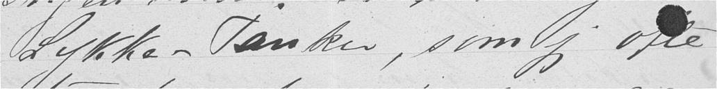Lykke-Tanker, som jeg ofte
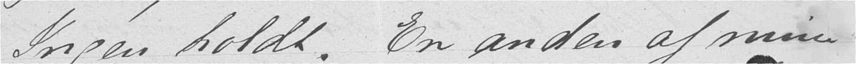Ingen holdt. En anden af mine
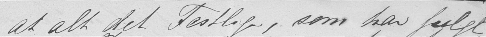at alt det Festlige, som har fulgt
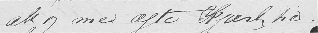alt og med ægte Kjærlighed.
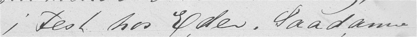i Fest hos Eder. Saadanne
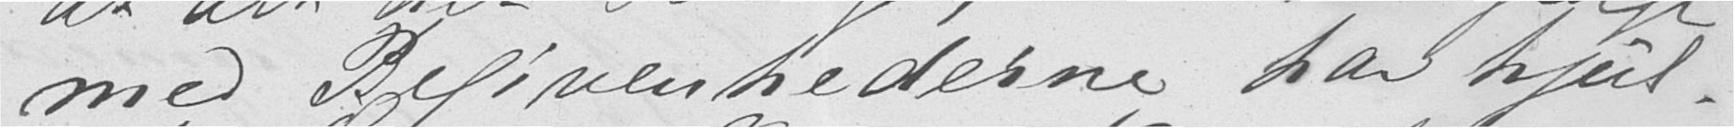med Begivenhederne har hjul-
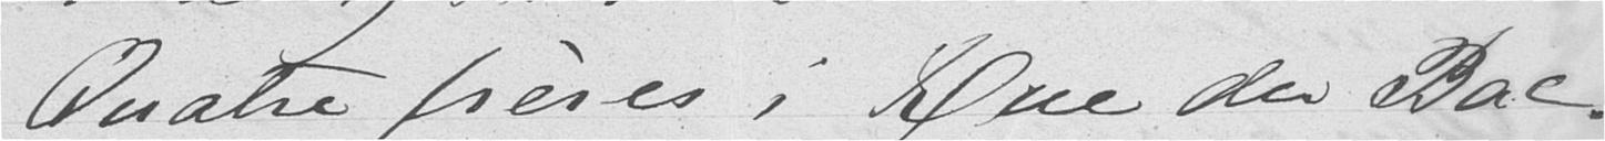Quatre frères i Rue der Bac.
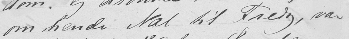om hende Nat til Fredag, var
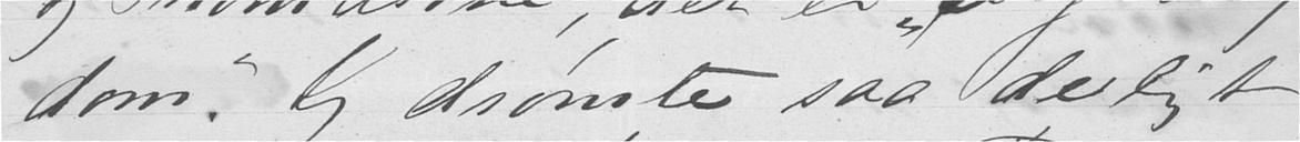dom". Jeg drømte saa deiligt
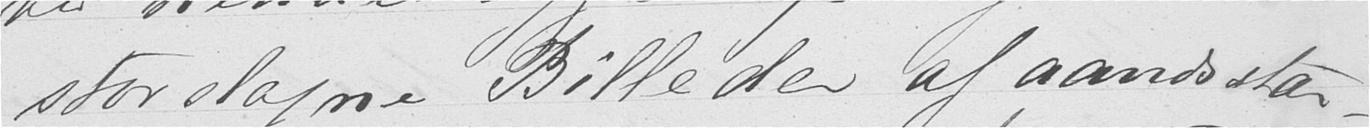storslagne Billeder af aandestær-
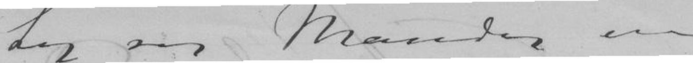tog sig Mandag en
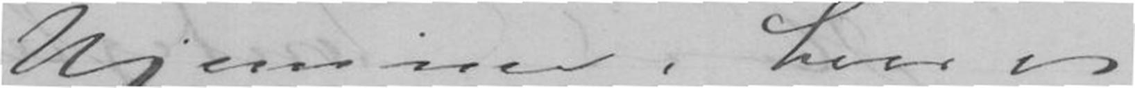Hjemme, hvor vi
